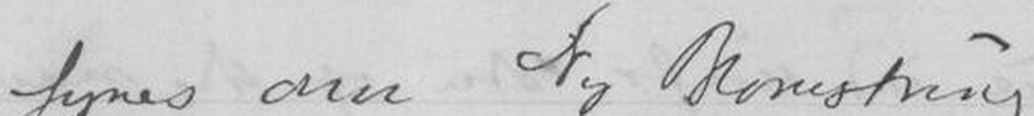Dig synes den Ny Blomstring
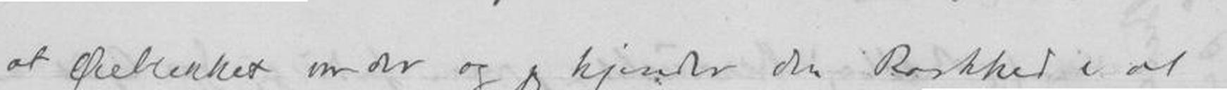at Øieblikket er der og jeg kjender din Raskhed i at
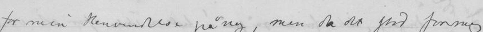for min Henvendelse på ny, men da det stod for mig
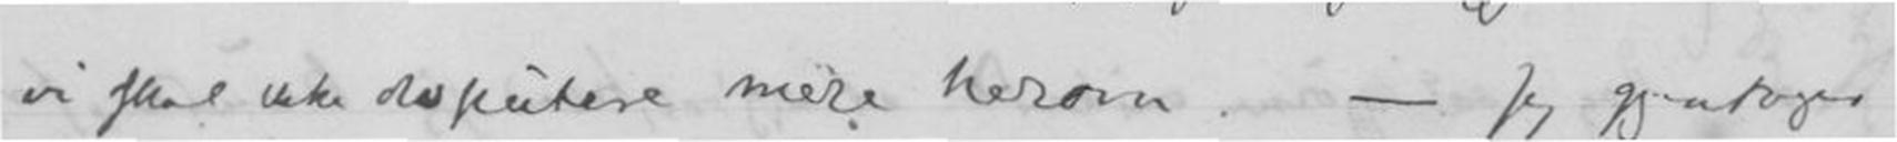vi skal ikke diskutere herom. - Jeg gjentager
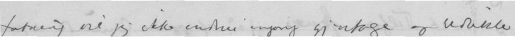fatning vil jeg ikke endnu engang gjentage og udvikle
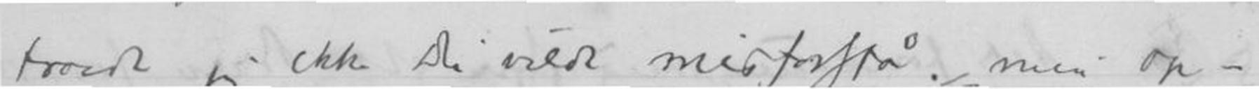troede jeg ikke Du vilde misforstå. men op-
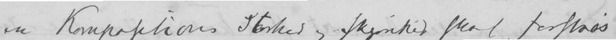en Kompositions storhed og skjønhed skal forståes.
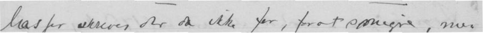has for skriver der da ikke for, for at smigre, men
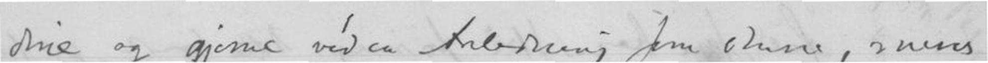dine og gjerne ved en Anledning som disse, snares
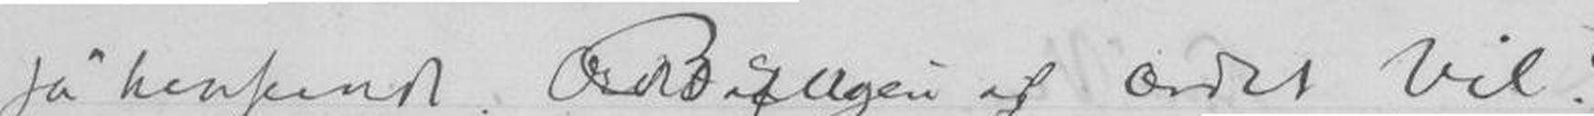i så henseende. Ord..åtllgen af Ordet vil?
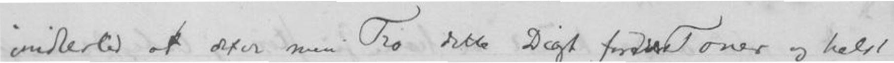imidlertid at det er min Tro dette Digt for disse Toner og helst
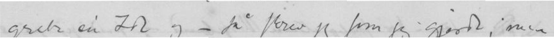gribe en Ide og så skrev jeg som jeg gjorde, men
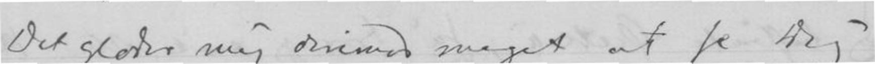Det glæder mig dermed meget at se
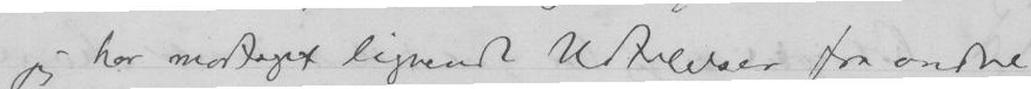jeg har modtaget lignende Udtalelser fra andre
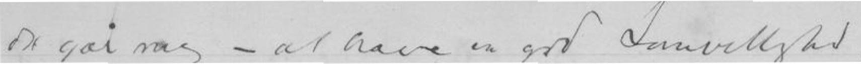det går rag - at have en god Sanvittighed
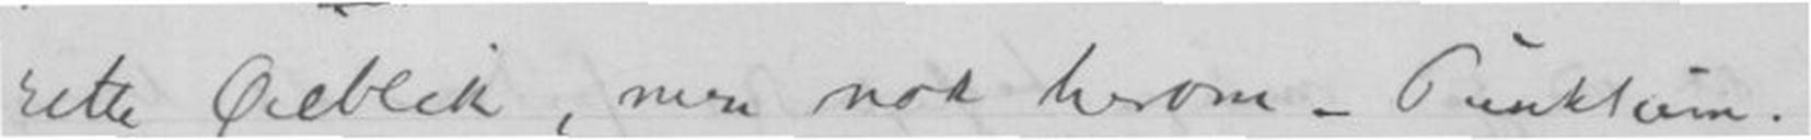rette Øieblik, men nok herom - Publikum.
